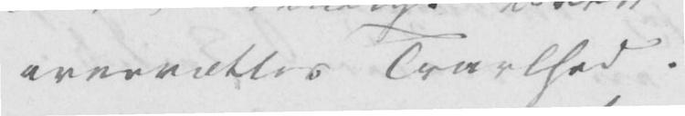overvættes Travlhed.
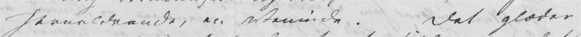Jevnaldrende, en Veninde. Det glæder
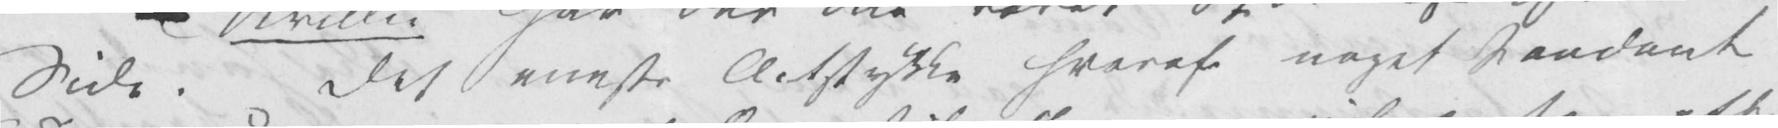Side. Det eneste Actstykke hvoraf noget Saadant
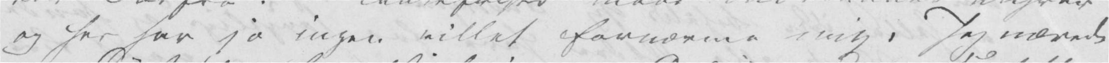og her har jo ingen villet fornærme mig. Jeg nærede
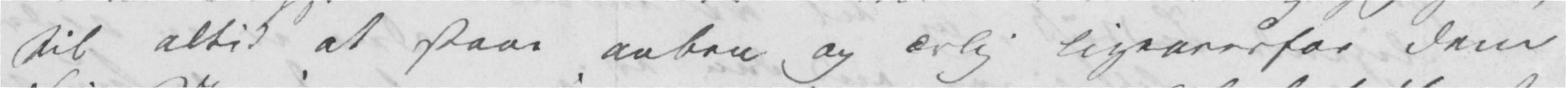til altid at staae aaben og ærlig ligeoverfor dem
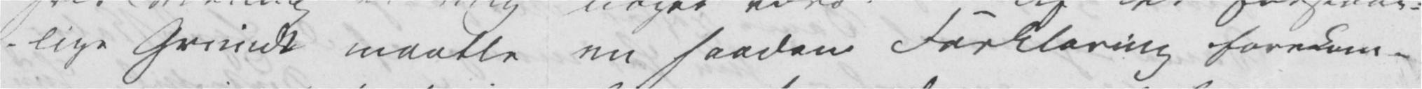lige Grunde maatte en saadan Forklaring forekom-
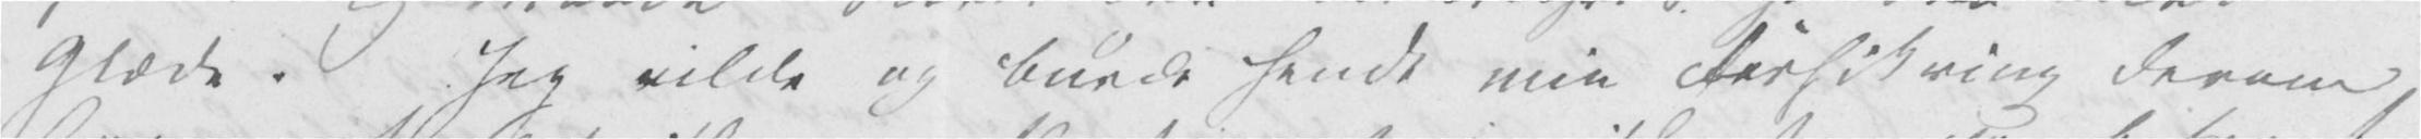Glæde. Jeg vilde og burde sendt min Forsikring derom
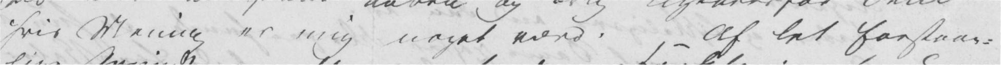hvis Mening er mig noget værd. Af let forstaae-
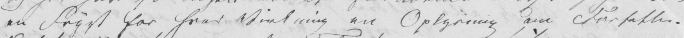en Frygt for hvad Virkning en Oplysning om Forfatter-
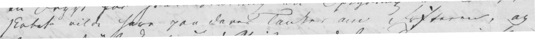skabet vilde have paa deres Tanker om Frfteren, og
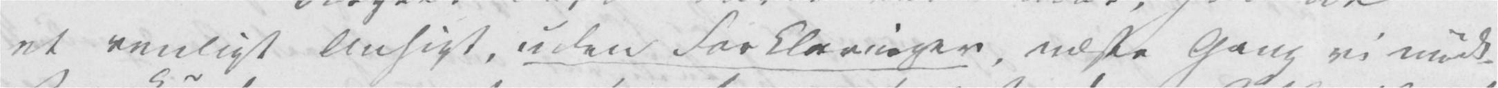et venligt Ansigt, uden Forklaringer, næste Gang vi mødtes
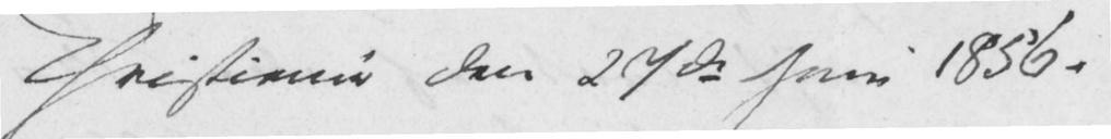Christiania den 27de Juni 1856.
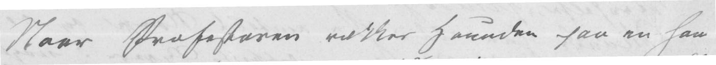Naar Professoren rækker Haanden paa en saa
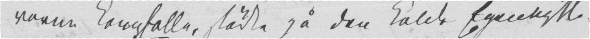varm Kampfælle, stødte på den kolde Egennytte.
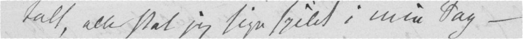talt, eller skal jeg sige spildt i min Sag –
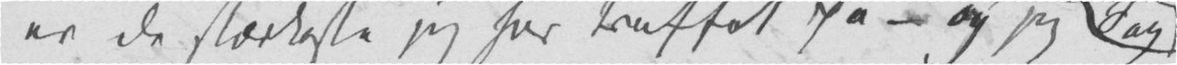er de stærkeste jeg har truffet på – og jeg Sag
